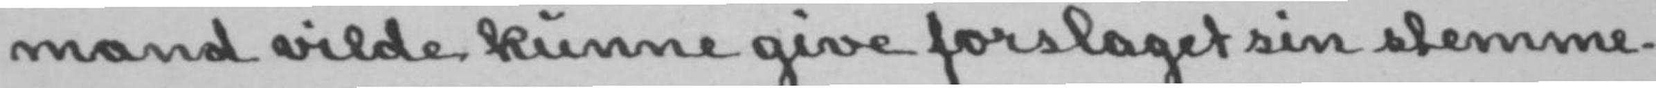mand vilde kunne give forslaget sin stemme.
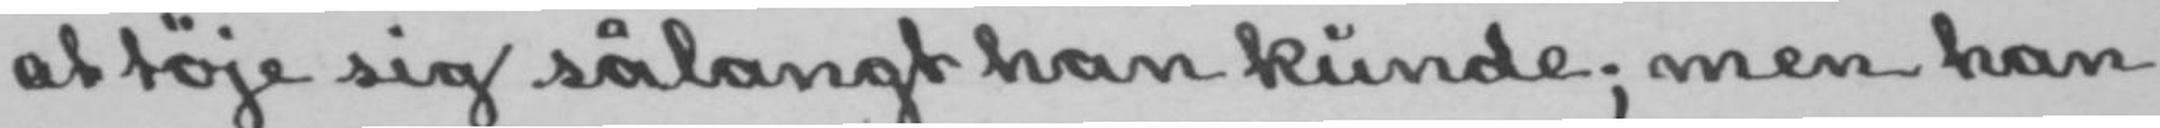at tøje sig sålangt han kunde; men han
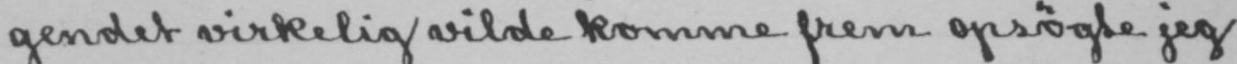gendet virkelig vilde komme frem opsøgte jeg
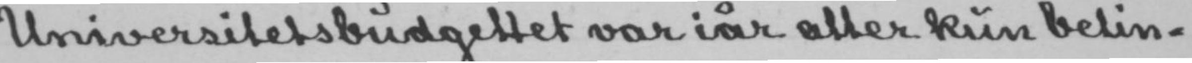Universitetsbudgettet var iår atter kun betin-
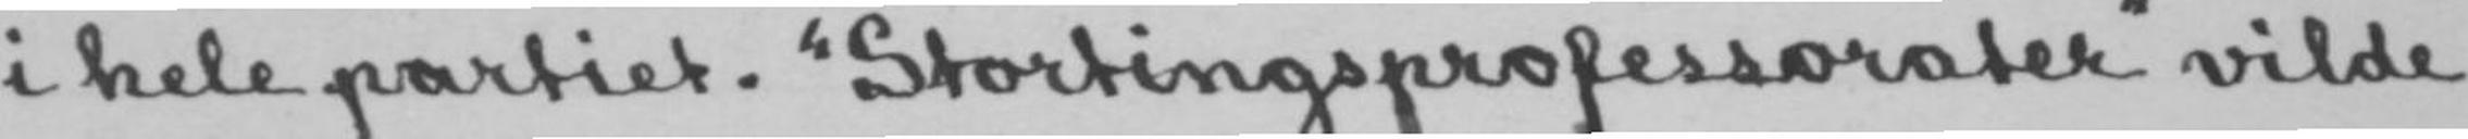i hele partiet. «Stortingsprofessorater» vilde
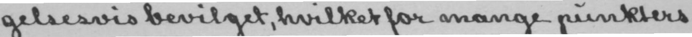gelsesvis bevilget, hvilket for mange punkters
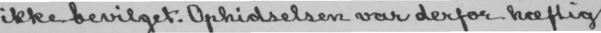ikke-bevilget. Ophidselsen var derfor hæftig
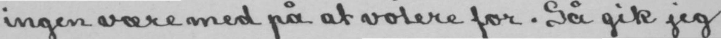ingen være med på at votere for. Så gik jeg
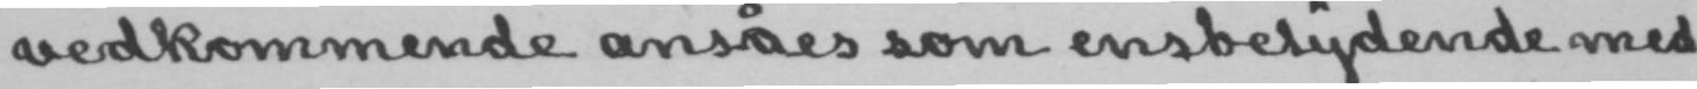vedkommende ansåes som ensbetydende med
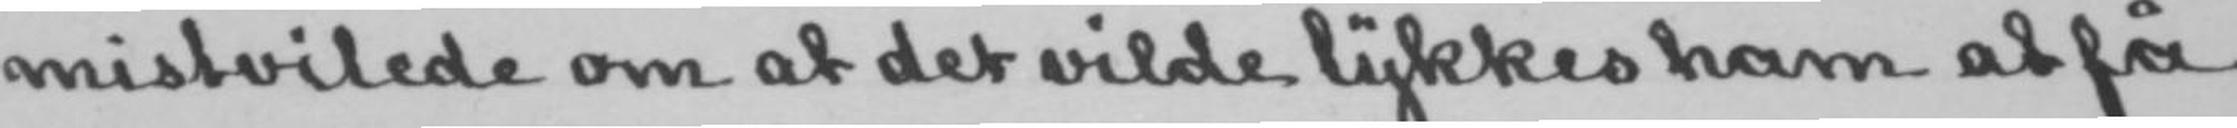mistvilede om at det vilde lykkes ham at få
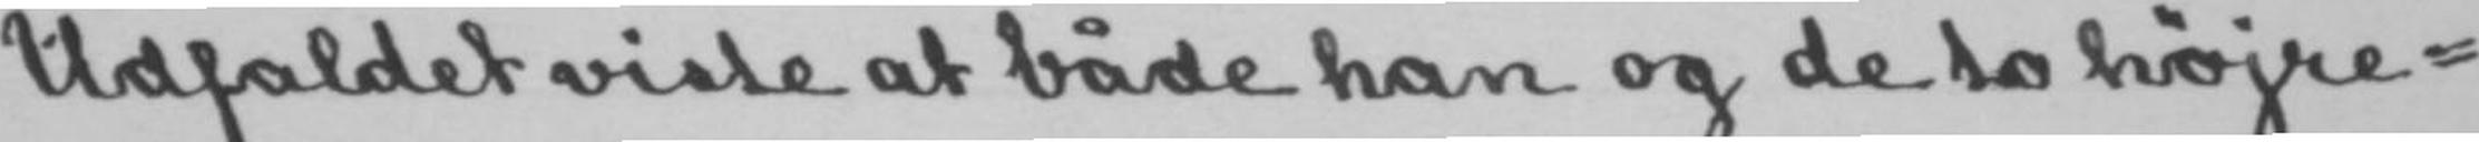Udfaldet viste at både han og de to højre-
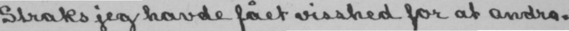Straks jeg havde fået visshed for at andra-
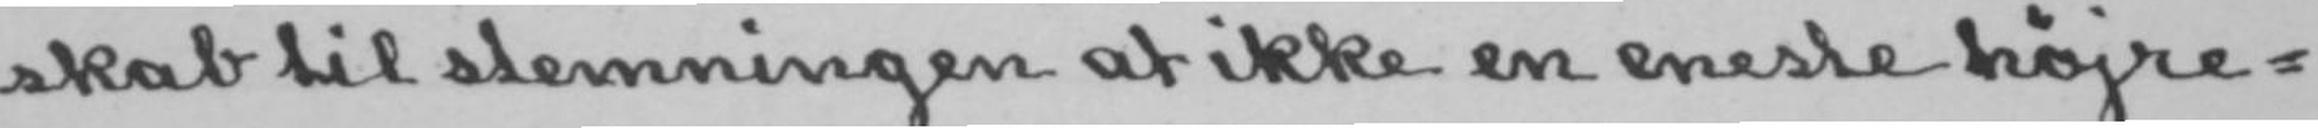skab til stemningen at ikke en eneste højre-
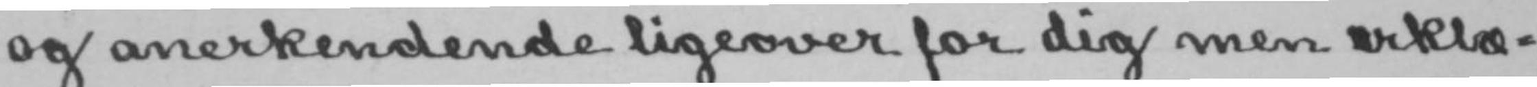og anerkendende ligeover for dig men erklæ-
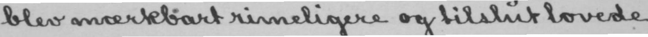blev mærkbart rimeligere og tilslut lovede
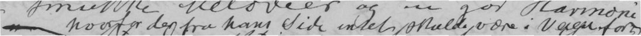hvorfor der fra hans Side intet skulde være i Vegen for
